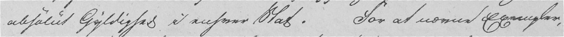absolut Gyldighed i enhver Stat. For at nævne Exempler,
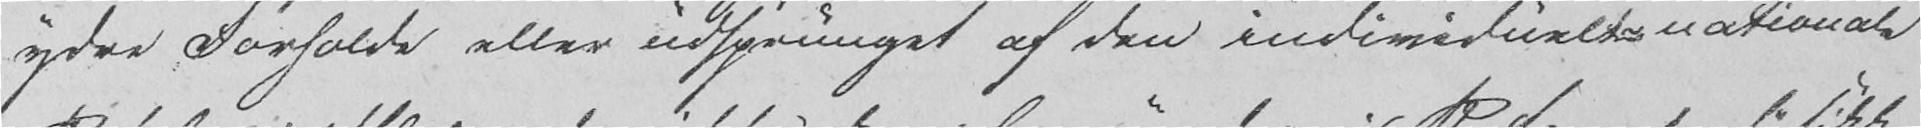ydre Forholde aller udsprunget af den individual-nationale
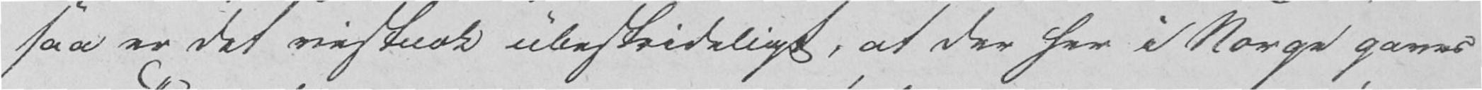saa er det vistnok ubestrideligt, at der her i Norge gaves
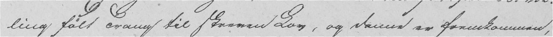ling følt Trang til skreven Lov, og denne er fremkommen,
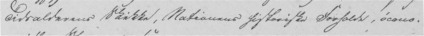Tidsalderens Skikke, Nationens historiske Forholde, øcono-
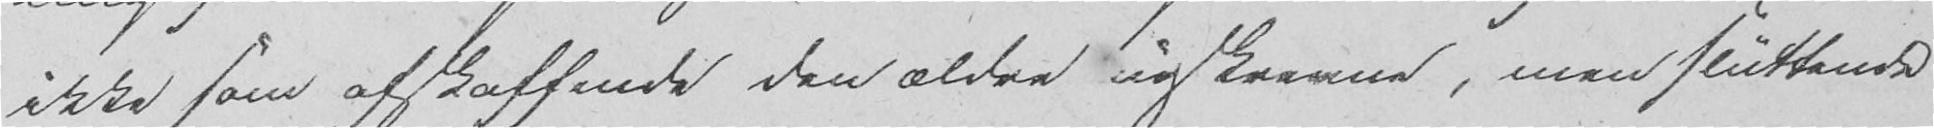ikke som afskaffende den ældre uskrevne, men sluttende
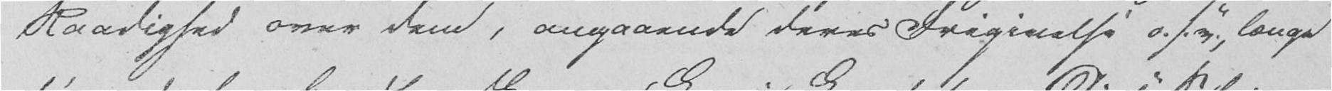Raadighed over dem, angaaende deres Frigivelse o. s. v., længe
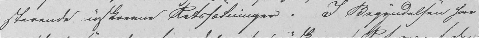sterende inskrevne Retssætninger. I Begyndelsen har
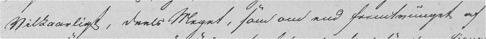Vilkaarligt, deels Meget, som om end fremtvunget af
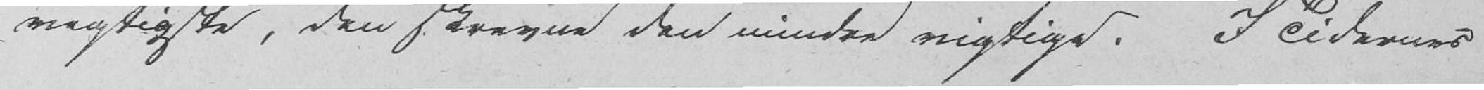vigtigste, den skrevne den mindre vigtige. I Tidernes
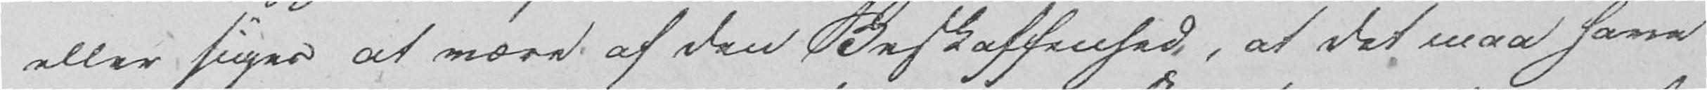eller siges at være af den Beskaffenhed, at det maa have
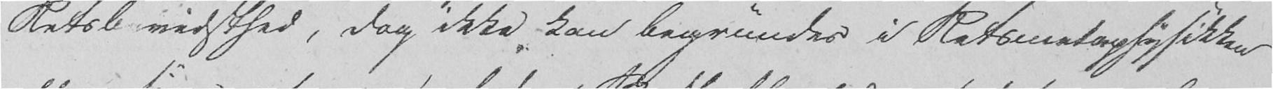Retsbevidsthed, dog ikke kan begrundes i Retsmetaphysikken
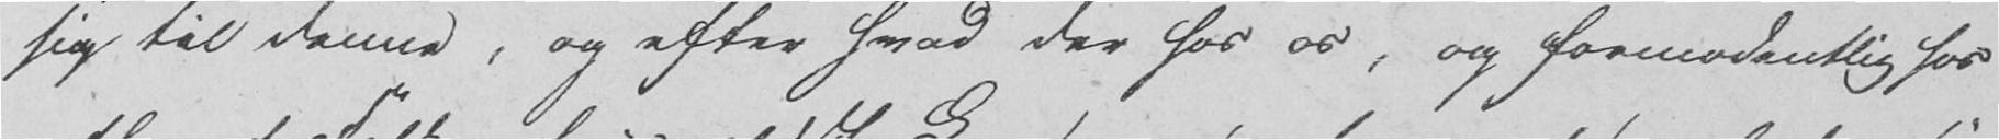sig til denne, og efter hvad der hos os, og formodentlig for
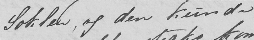Soklen, og den kunde
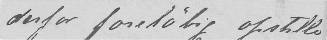derfor foreløbig opstille
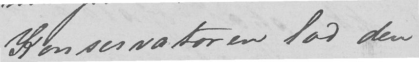Konservatoren lod den
